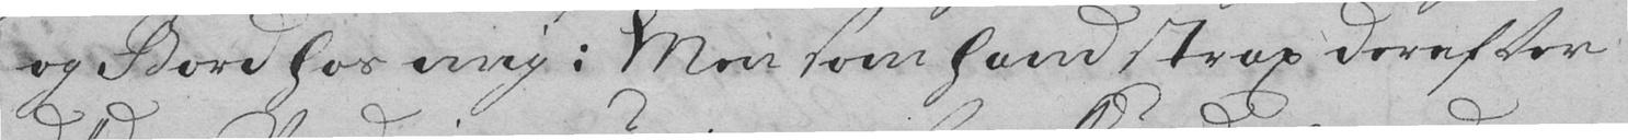og Bord hos mig; Men som hand strax derefter
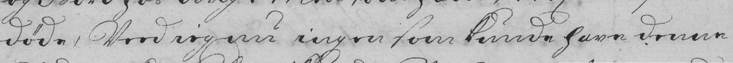døde, Veed ieg nu ingen som kunde have denne
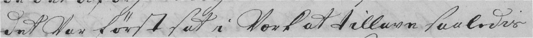det var først sat i Værk at tillave saaledis
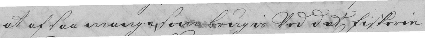at af saa mange, som brugis Ved det Fiskerie
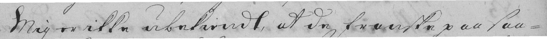Mig er ikke ubekiendt, at de Franske paa saa-
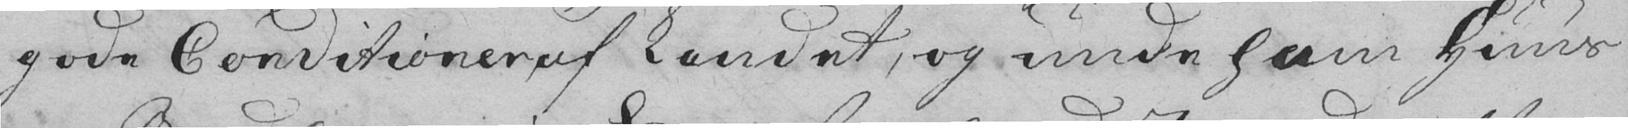gode Conditioner af Landet, og unde ham Huus
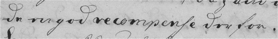de en god recompense der for.
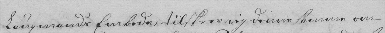Laugmands Embede, tilskrev sig denne samme om
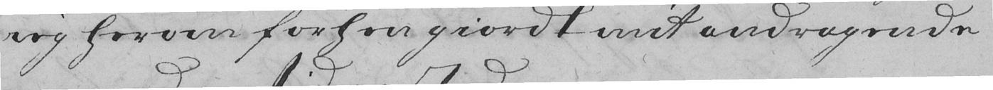ieg herom forhen giordt mit andragende
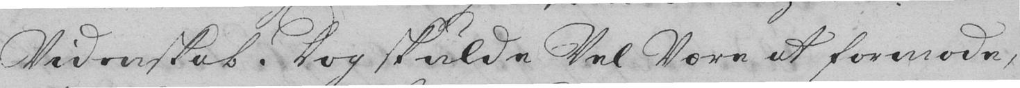Videnskab. Dog skulde Vel Være at formode,
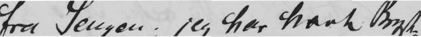fra Sengen; jeg har havt Bryst-
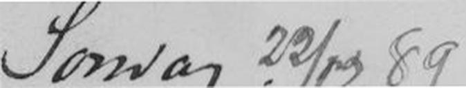Sondag 22/12 89
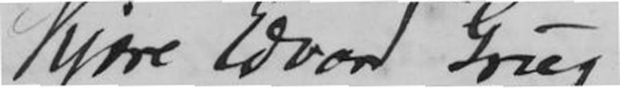Kjære Edvard Grieg!
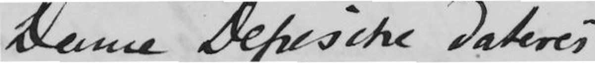Denne Depische dateres
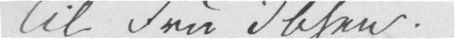Til Fru Ibsen!
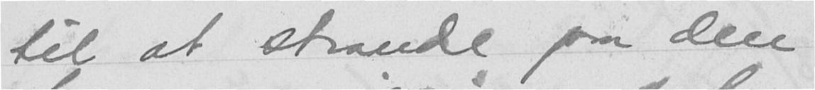til at strande paa den
